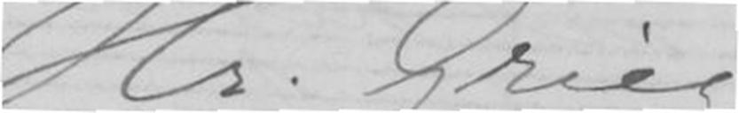Hr. Grieg
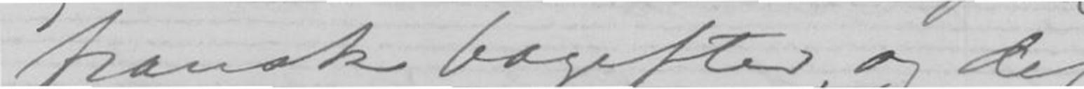fransk bagefter, og det
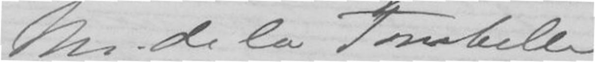Mr. de la Tombelle
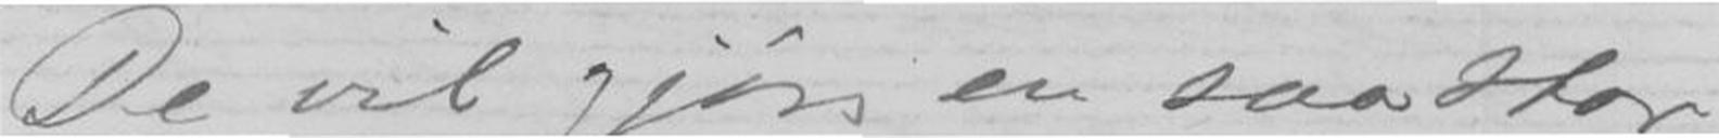De vil gjøre en saa stor
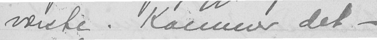værste. Kommer det -
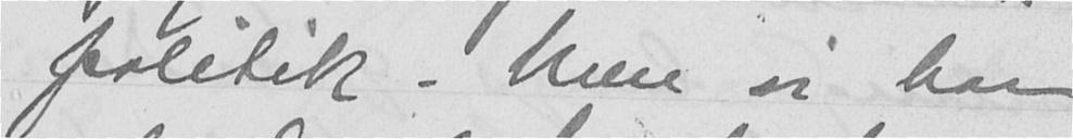politik. Men vi har
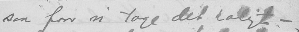saa faar vi tage det roligt -
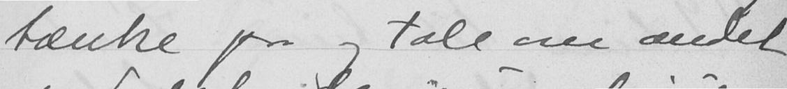tænke paa og tale om andet
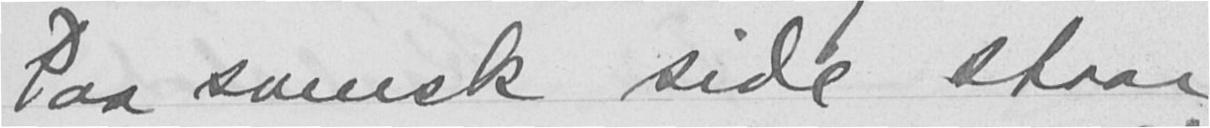Paa svensk side staar
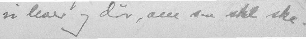vi lever og dør, om saa skal ske.
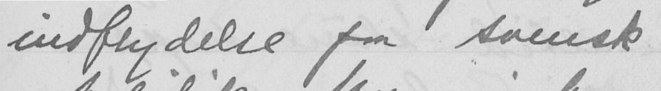indflydelse paa svensk
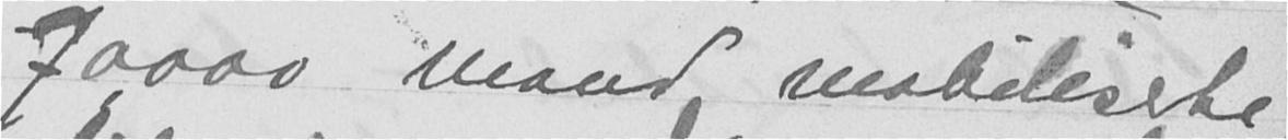70000 mand mobiliserte,
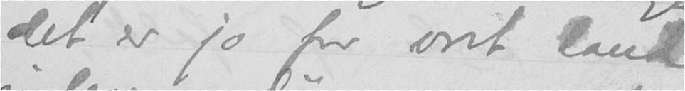det er jo for vort land
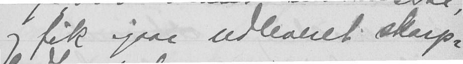og fik igaar udleveret skarp-
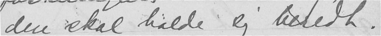den skal holde sig beredt.
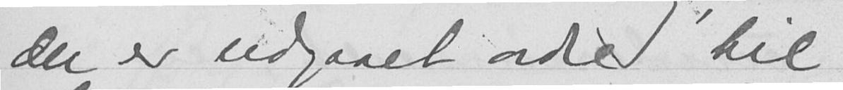der er udgaaet ordre til
